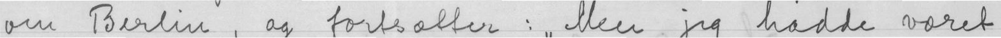om Berlin, og fortsætter: "Men jeg hadde været
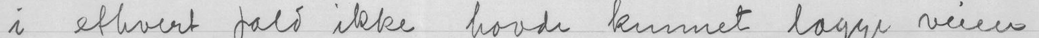i ethvert fald ikke havde kunnet lægge veien
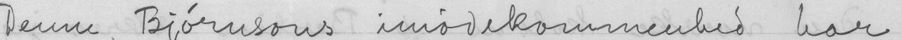Denne Bjørnsons imødekommenhed har
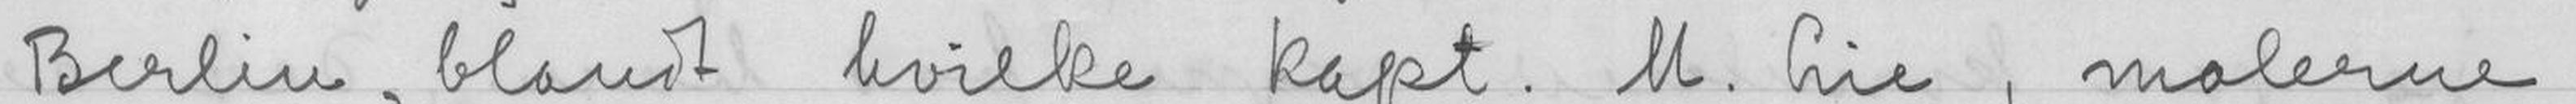Berlin, blandt hvilke kapt. Lie, maleren
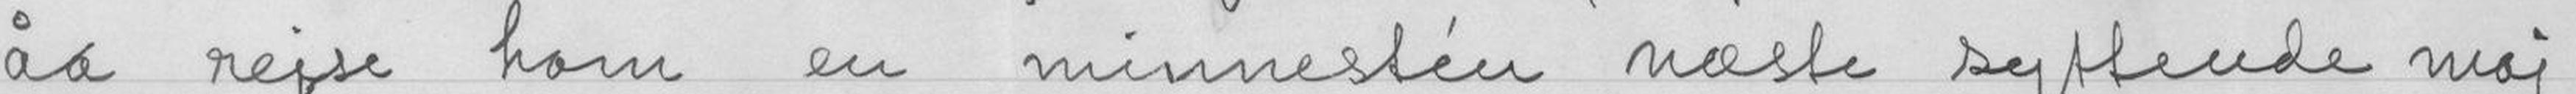åa rejse ham en minnestén næste syttende maj
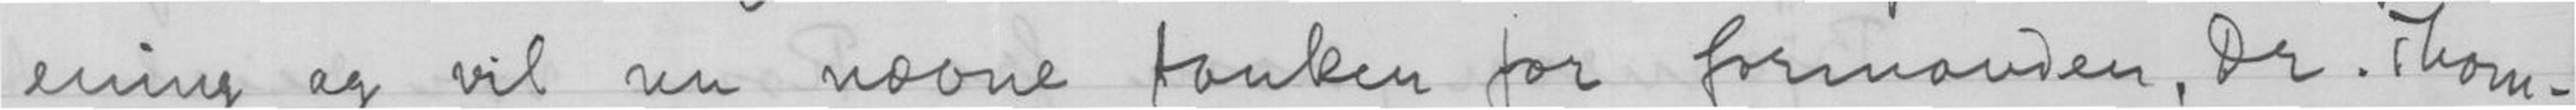ening og vil nu nævne tanken for formanden, Dr Thom-
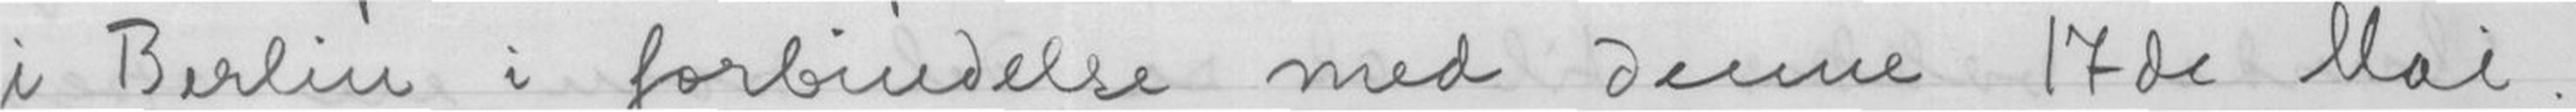i Berlin i forbindelse med denne 17de Mai.
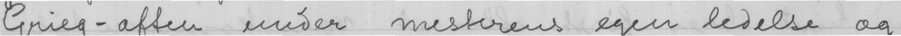Grieg-aften under mesterens egen ledelse og
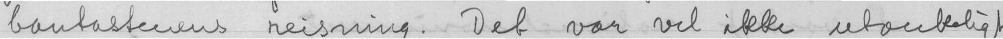bautastenens reisning. Det var vel ikke utænkeligt
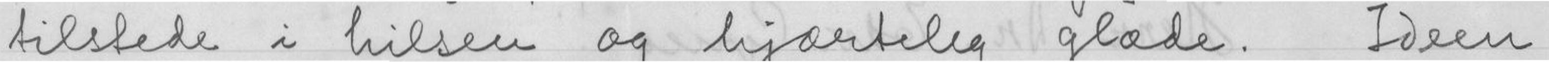tilstede i hilsen og hjærtelig glæde. Ideen
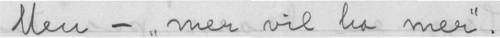Men - "mer vil ha mer".
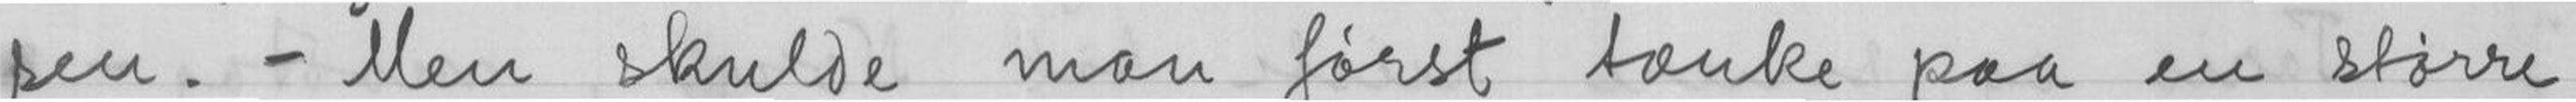sen. - Men skulde man først tænke paa en større
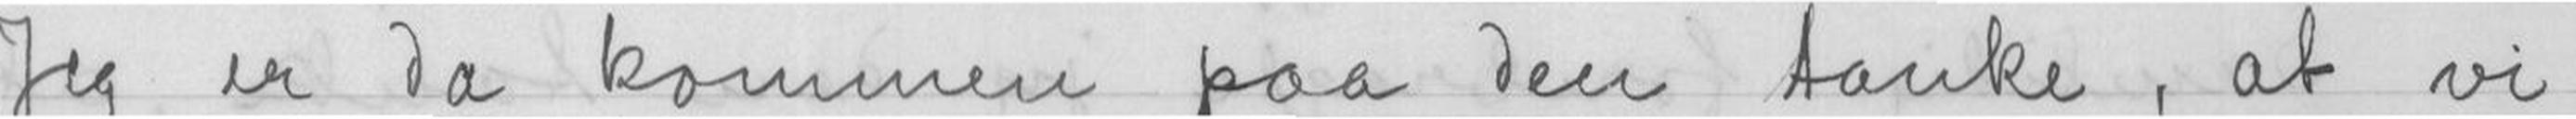Jeg er da kommen paa den tanke, at vi
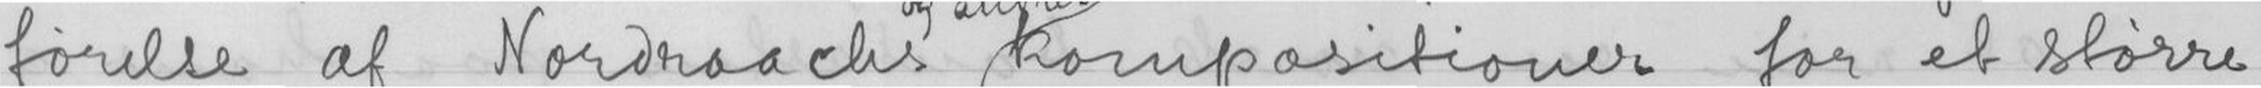førelse af Nordraachs og andres kompositioner for et større
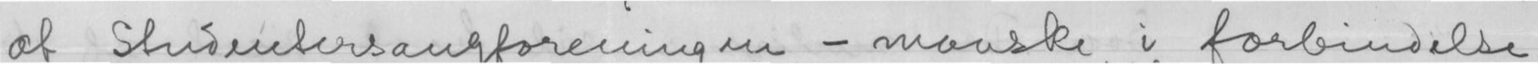at Studentersangforeningen - maaske i forbindelse
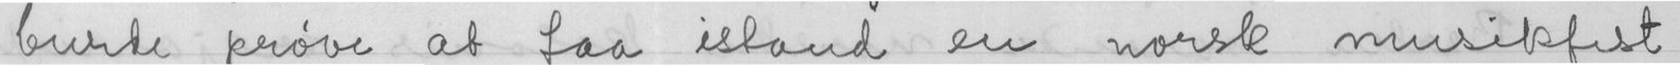burde prøve at faa istand en norsk musikfest
